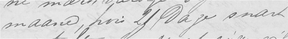maaned, hvis 21 Dage snart
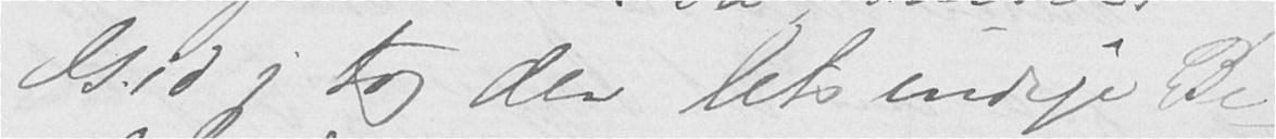Gid jeg tog den letsindige Be-
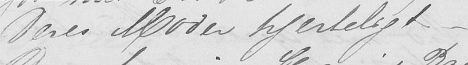Deres Moder hjerteligt -
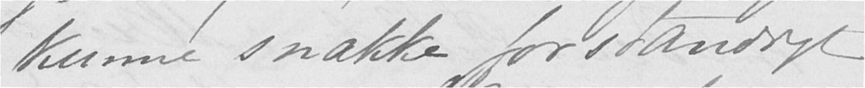kunne snakke forstandigt
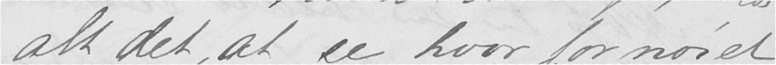alt det, at se hvor fornøiet
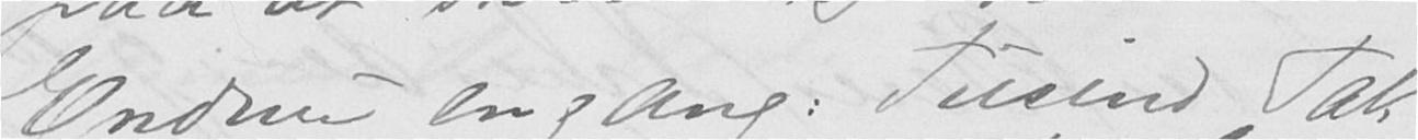Endnu engang: Tusind Tak
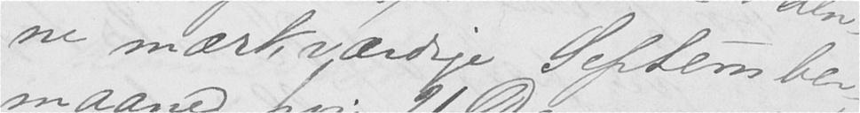ne mærkværdige September-
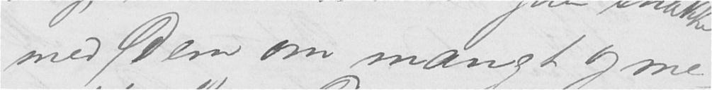med Dem om mangt og me-
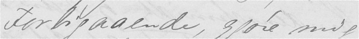Forbigaaende, gjøre mig
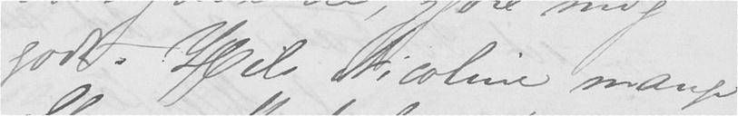godt. Hils Nicoline mange
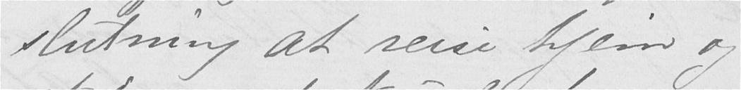slutning at reise hjem og
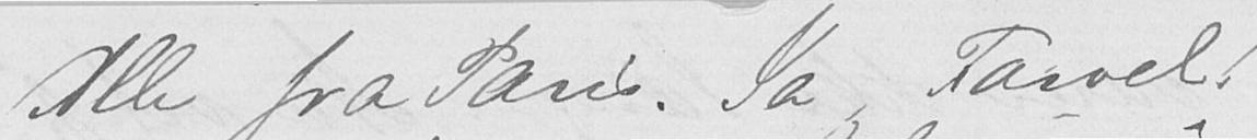alle fra Paris. Ja, Farvel!
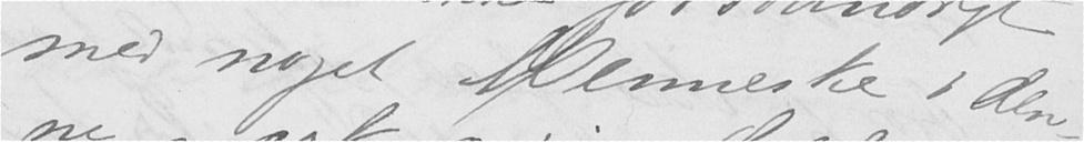med noget Menneske i den-
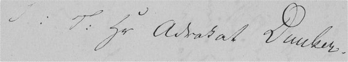S: T: Hr Advokat Dunker.
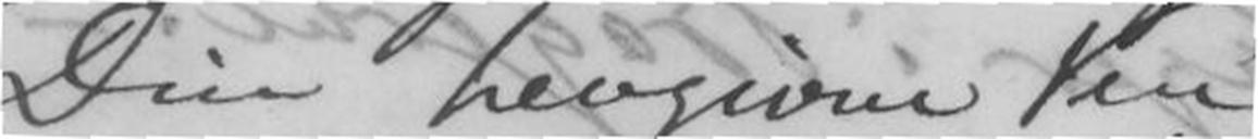Din hengivne Ven
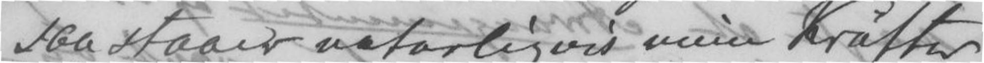saa staaer naturligvis mine Kræfter
